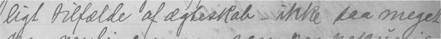ligt tilfælde af ægteskab - ikke saa meget
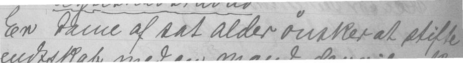En dame af sat alder ønsker at stifte
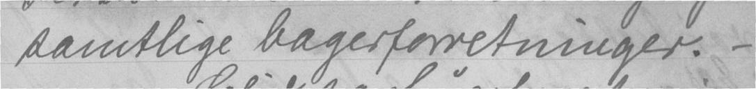samtlige bagerforretninger. -
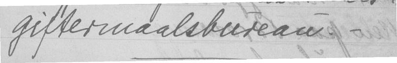giftermaalsbureau. -
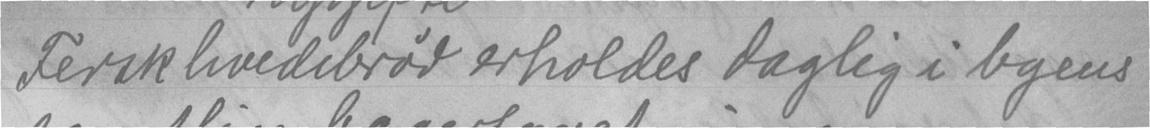Fersk hvedebrød erholdes daglig i byens
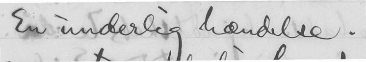En underlig hændelse.
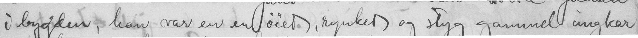i bygden, han var en en øiet, rynket og styg gammel ungkar
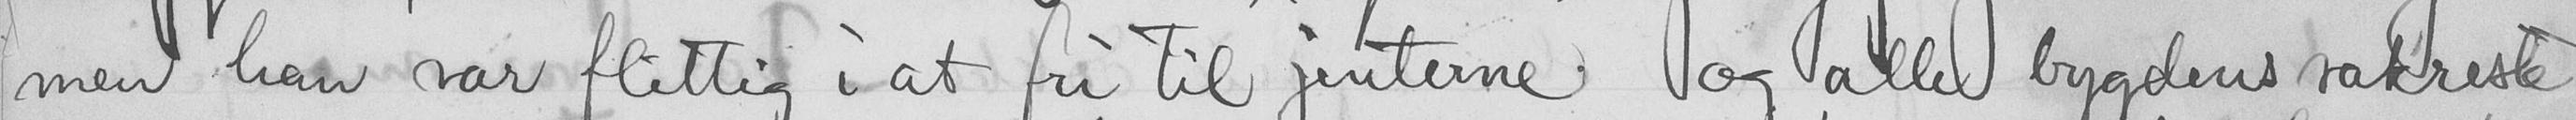men han var flittig i at fri til jenterne og alle bygdens vakreste
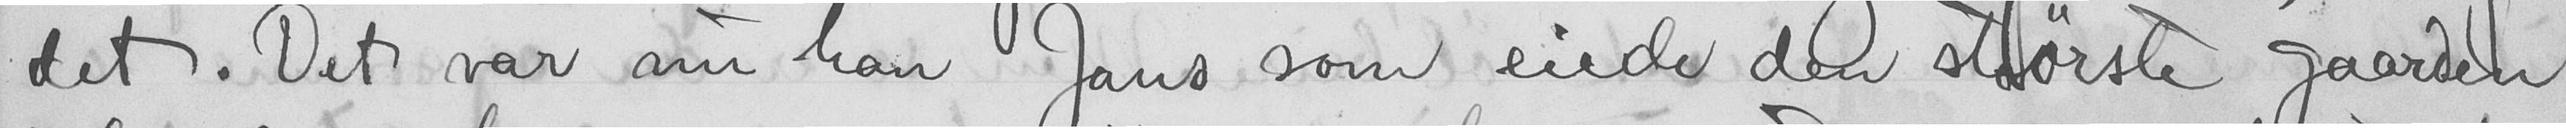det. Det var nu han Jans som eiede du største gaarden
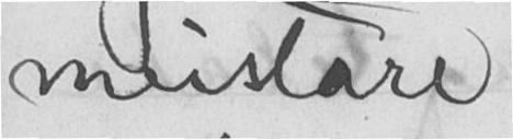meistare
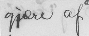gjære af
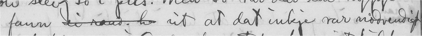fann  ut at dat inkje var nødvendigt
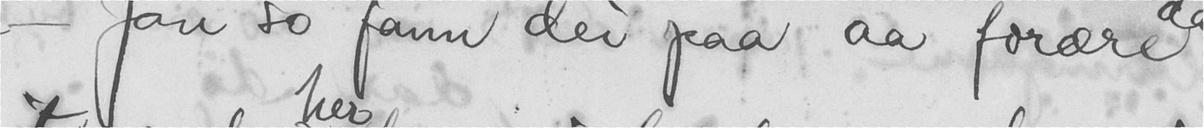- Jau so fann dei paa aa forære vær i Solei
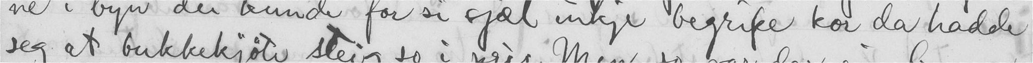ne i byn dei kunde for si sjæl inkje begripe kor da hadde
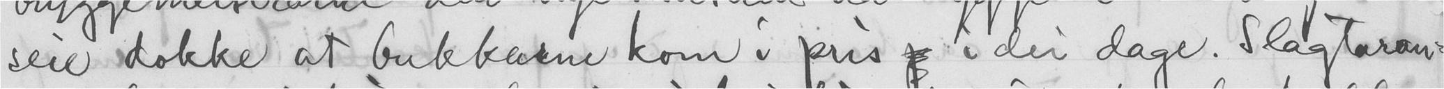seie dokke at bukkurne kom i pris  i dei dage. Slagtaran-
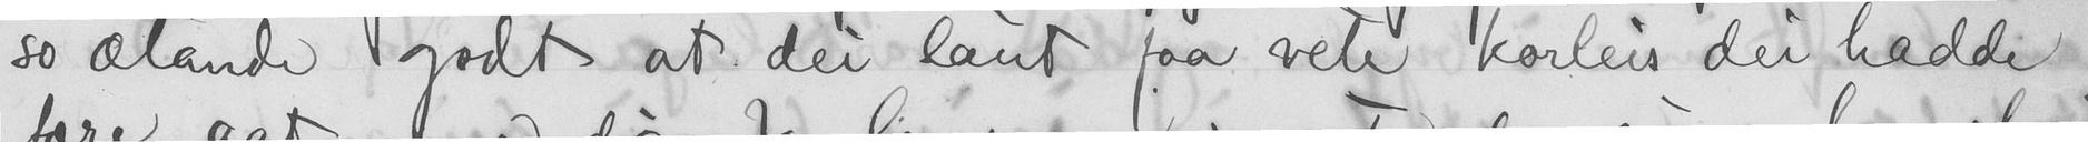so ætande godt at der laut faa vete korleis dei hadde
